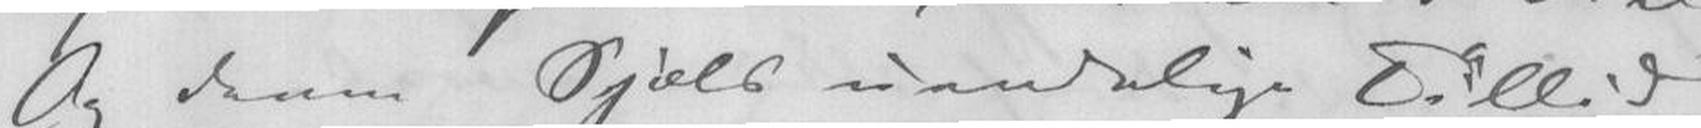Og denne Sjæls uendelige Tillid
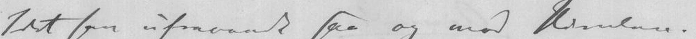I det han ufravandt saa op mod Himlen. -
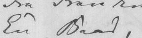En Baad,
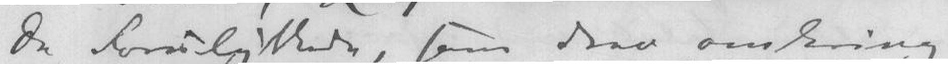De forulykkede, som drev omkring.
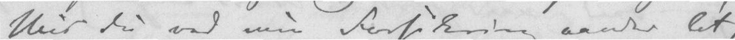Hvis du ved min Forsikring aander let,
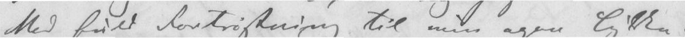Med fuld Fortrøstning til min egen Lykke;
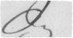Og
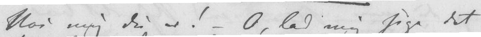Hos mig du er! - O, lad mig sige det
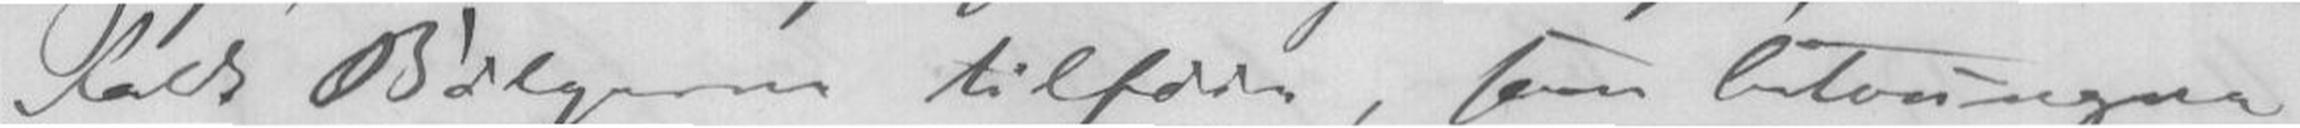Faldt Bølgerne tilside, som betvungne
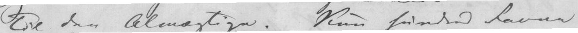Til den Almægtige. Kun hundred Favner
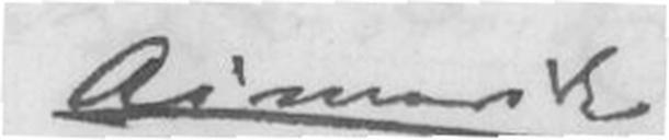Aimerik
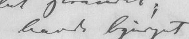havde bjerget
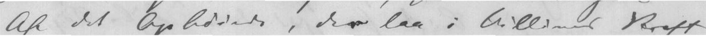Af det Ophøiede, som laa i Villines Kraft
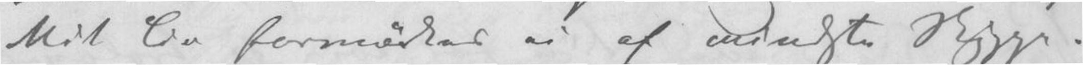Mit Liv formørker ei af mindste Skygge.
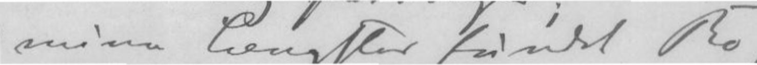mine Længsler fundet Ro,
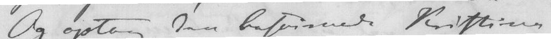Og optog den besvimede Kristina
